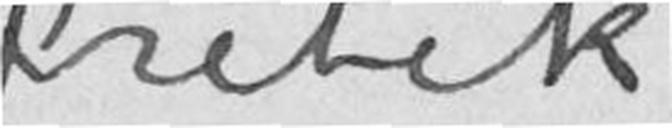kritik
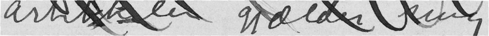artikkelen gjælder mig
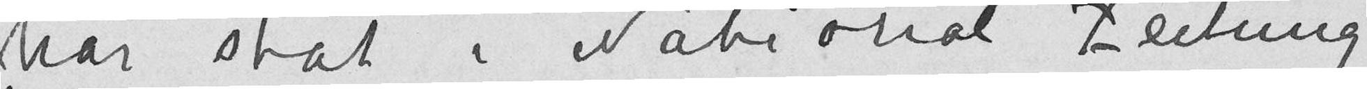har ståt i National Zeitung
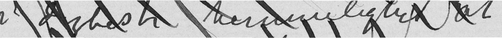i dybeste hemmelighed at
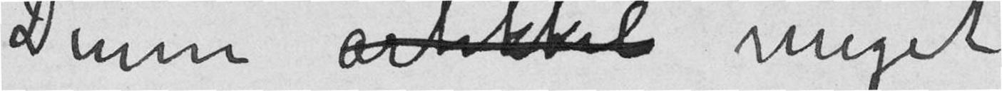Denne artikkel meget
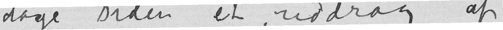dage siden et uddrag af
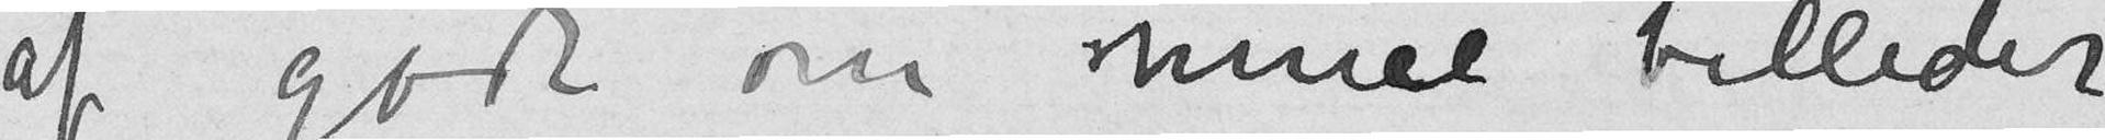af godt om mine billeder
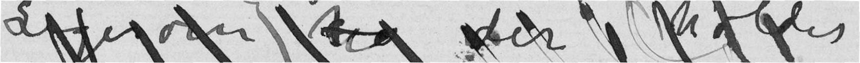Ligesom der holdes
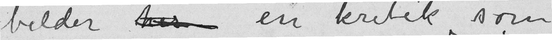bilder hos en kritik som
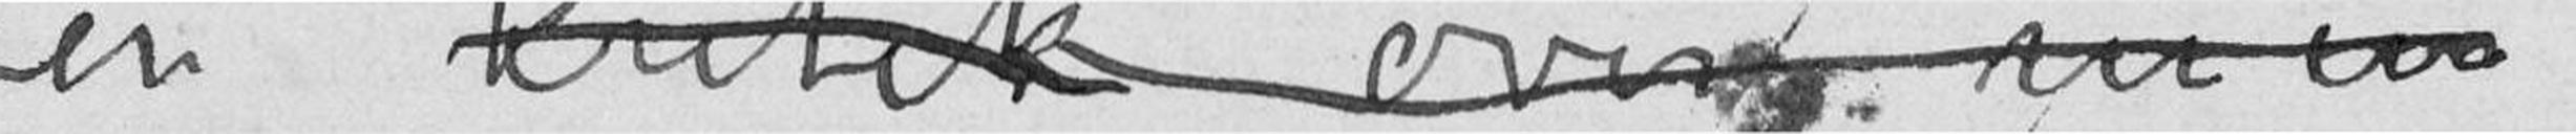en kritik over min
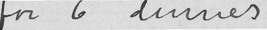for 6 dennes
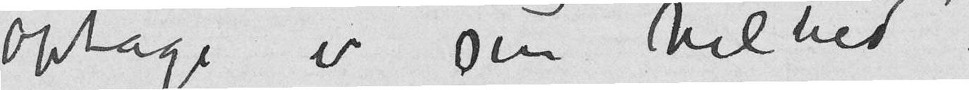optage i sin helhed –
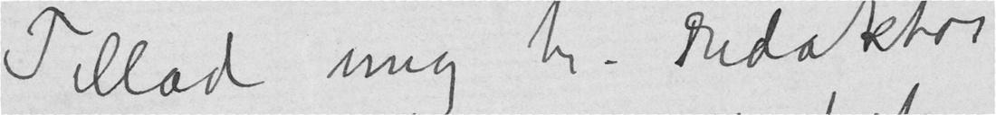Tillad mig hr. redaktør
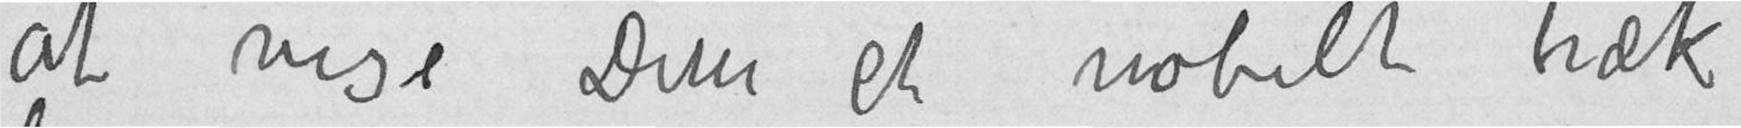at vise Dem et nobelt træk
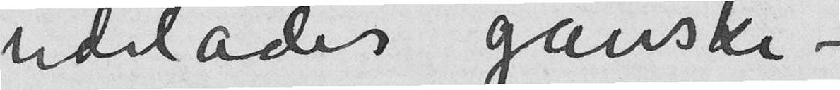udelades ganske –
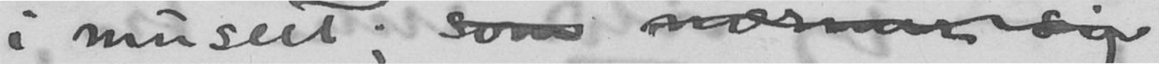i museet; som nærmer sig
